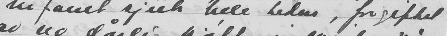infamt sjuk hele tiden, forgiftet
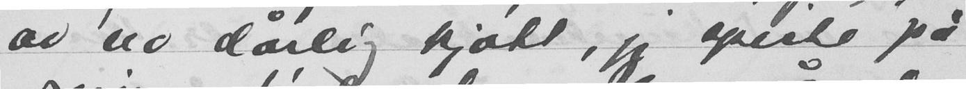av no dårlig kjøtt, jeg spiste på
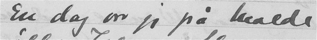Én dag var jeg på Molde
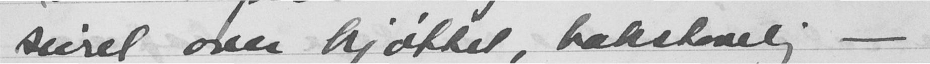seiret over kjøttet, bokstavelig -
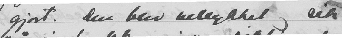gjort. Den blev vellykket og rik
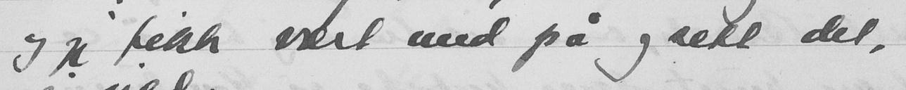og jeg fikk vært med på og sett det,
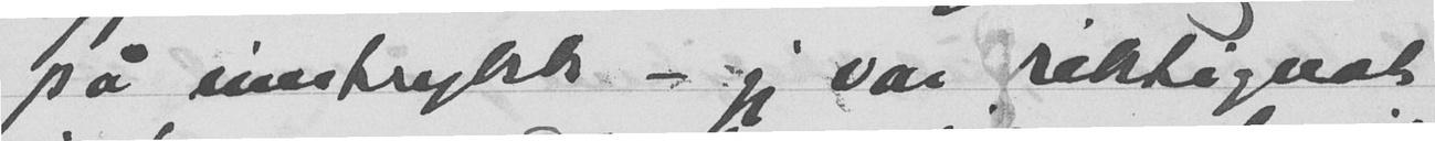på inntrykk - jeg var riktignok
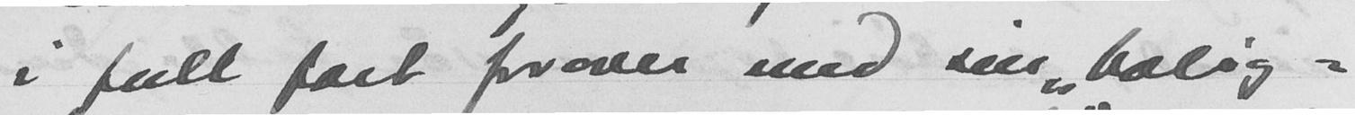i full fart forover med sin "bolig-
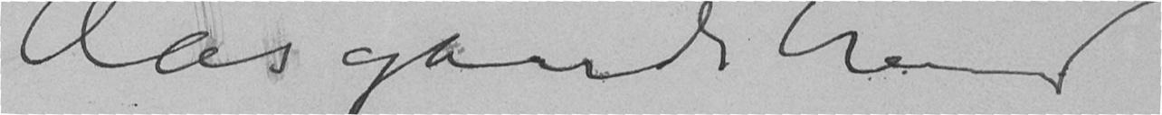Aasgaardstrand
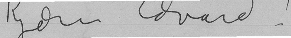Kjære Edvard!
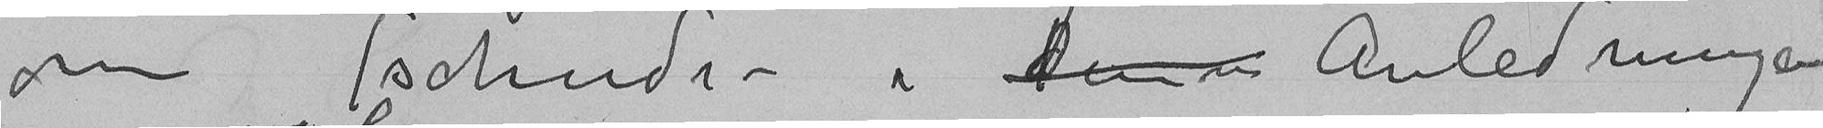om Tschudi i Anledningen
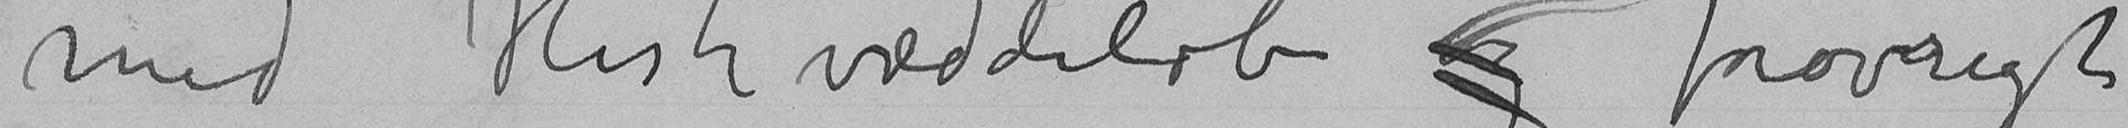med Hestevæddeløb og forøvrigt
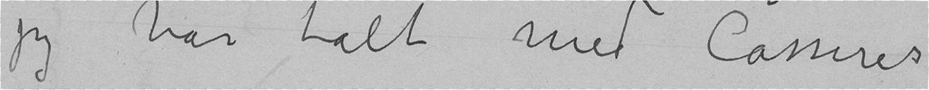jeg har talt med Cassirer
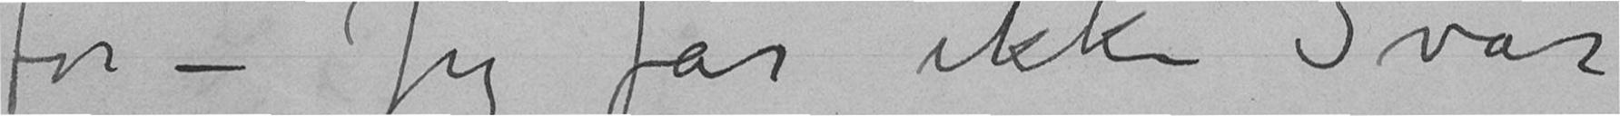for – Jeg får ikke Svar
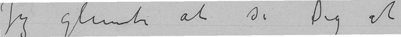Jeg glemte at si Dig at
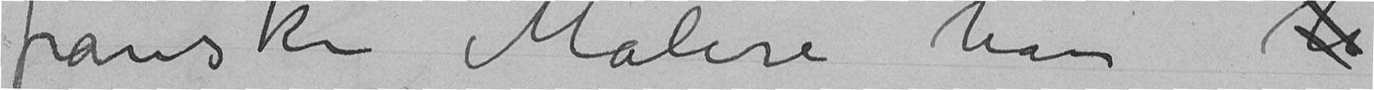franske Malere han h
